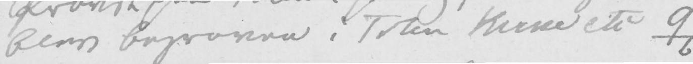blev begroven i Toten Kirke etc q
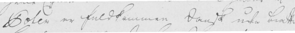Toten er fuldkommen Dansk uden bratte
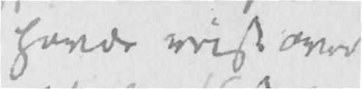havde reist over
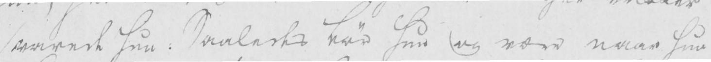svarede hun: Saaledes bør hun og være naar hun
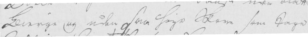Bierge og uden saa høye Skove som tage
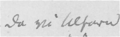da vi tilforn
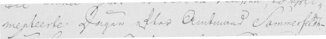menteerte Dagen efter Amtmand Sommerfeldt -
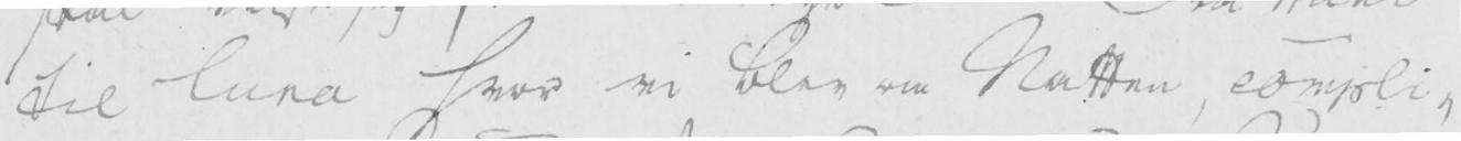til Lena hvor vi blev om Natten, compli-
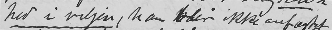hed i viljen, han blir ikke anfægtet
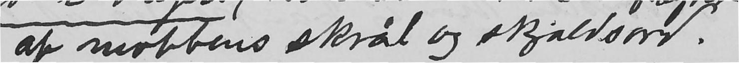af mobbens skrål og skjældsord.
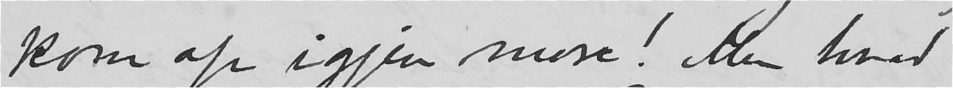kom op igjen mere! Men hvad
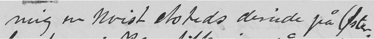mig en kvist etsteds derinde på Øster-
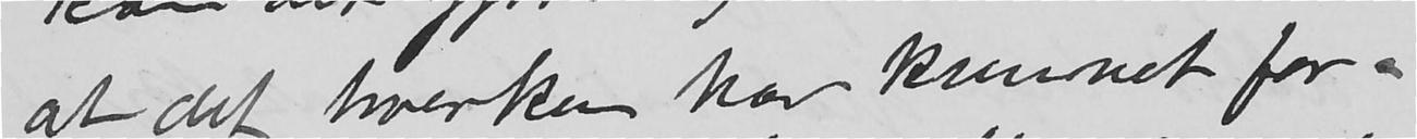at det hverken har kunnet for-
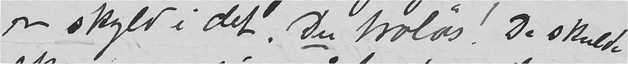er skyld i det. Du troløs! De skulde
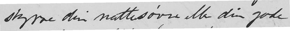styrre din nattesøvn elle din gode
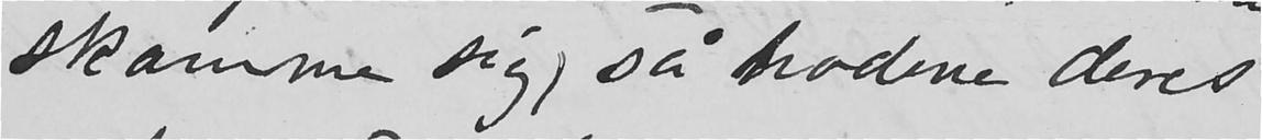skamme sig, så hodene deres
